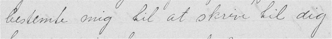bestemte mig til at skrive til dig —
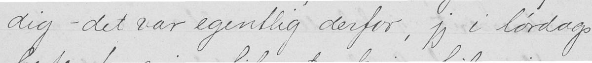dig — det var egentlig derfor, jeg i lørdags
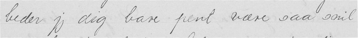beder jeg dig bare pent være saa snil
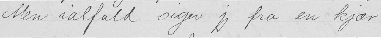Men ialfald siger jeg fra en kjær
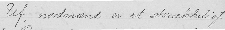Uf, nordmænd er et skrækkeligt
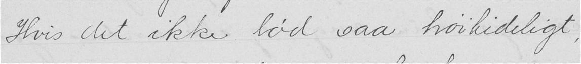Hvis det ikke lød saa høitideligt,
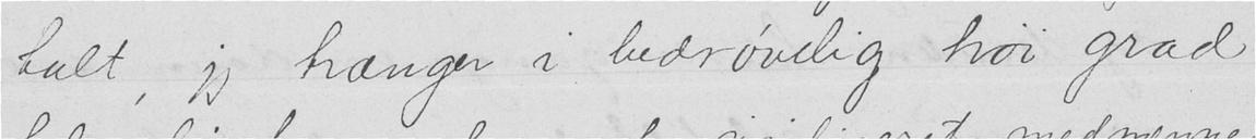talt, jeg trænger i bedrøvelig høi grad
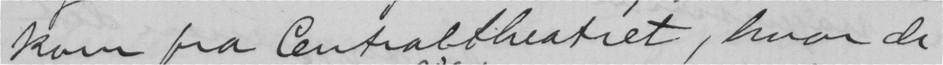kom fra Centraltheatret, hvor de
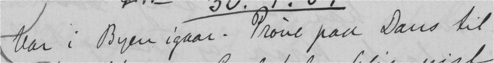Var i Byen igaar. Prøve paa Dans til
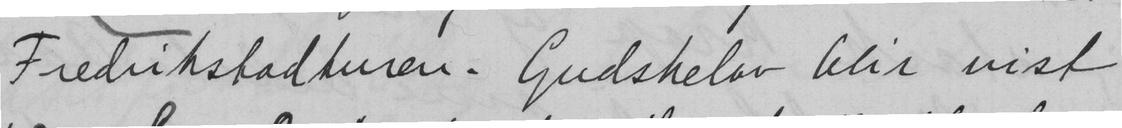Fredrikshaldturen. Gudskelov blir vist
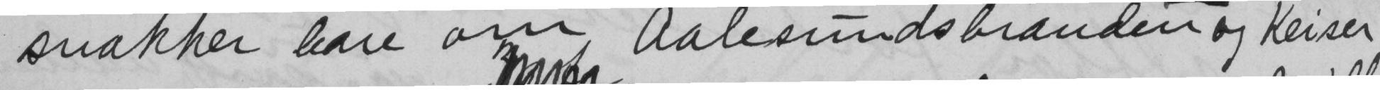snakker bare om Aalesundsbranden og Keiser
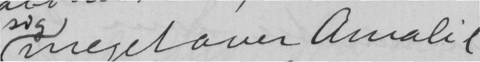meget over Amalie
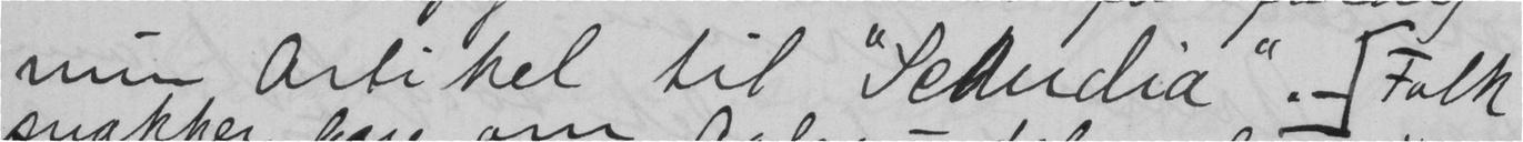min Artikel til "Scandia". Folk
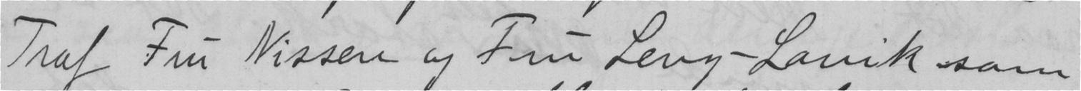Traf Fru Nissen og Fru Levy-Lavik som
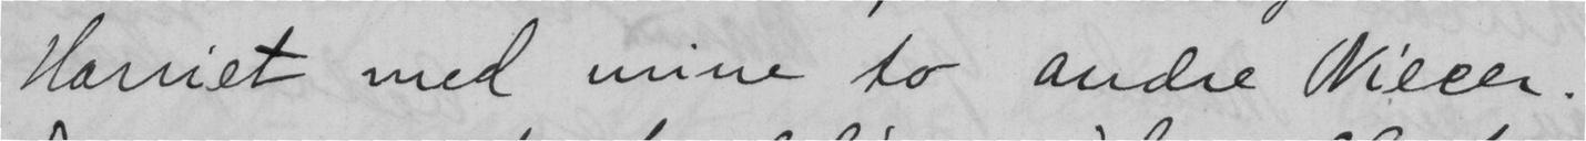Harriet med mine to andre Niecer.
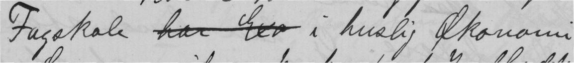Fagskole  i huslig Økonomi
